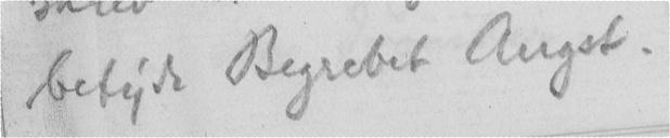betyde Begrebet Angst.
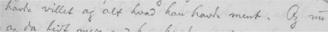havde villet og alt hvad han havde ment. Og nu
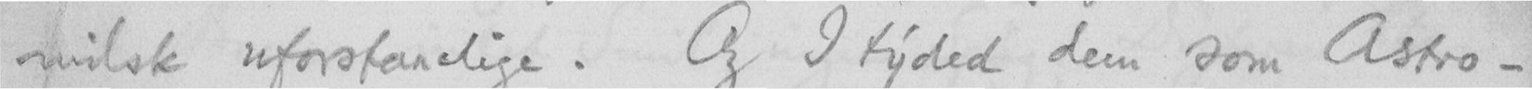milsk uforstaaelige. Og I tyded dem som Astro-
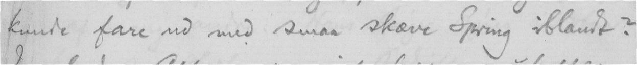kunde fare ud med smaa skæve Spring iblandt?
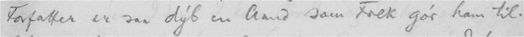Forfatter er saa dyb en Aand som Folk gør ham til.
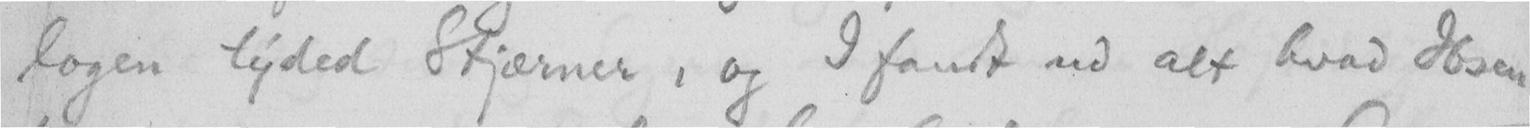logen tyded Stjærner, og I fandt ud alt hvad Ibsen
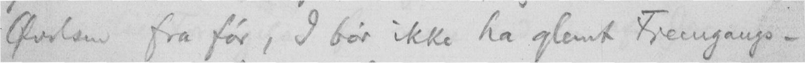Øvelsen fra før, I bør ikke ha glemt Fremgangs-
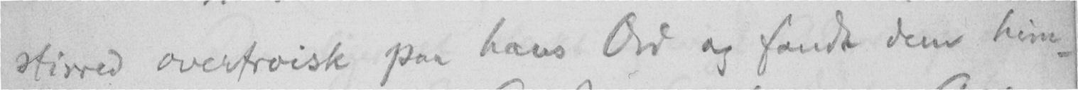stirred overtroisk paa hans Ord og fandt dem him-
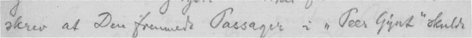skrev at Den fremmede Passager i "Peer Gynt" skulde
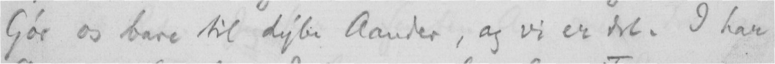Gør os bare til dybe Aander, og vi er det. I har
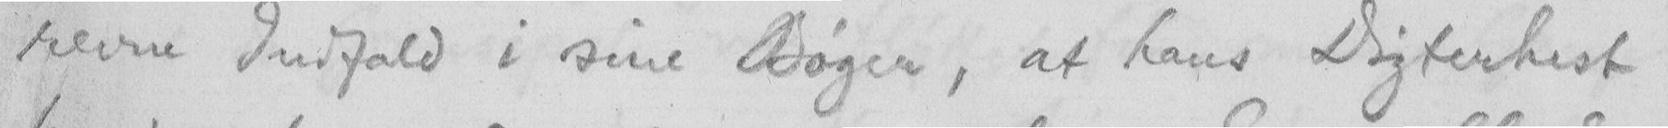revne Indfald i sine Bøger, at kans Digterhest
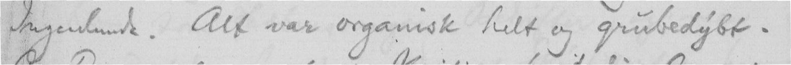Ingenlunde. Alt var organisk helt og grubedybt.
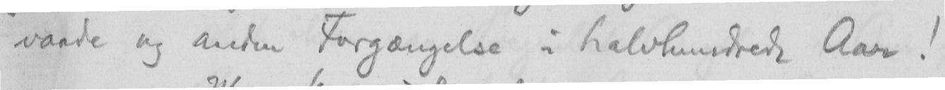vaade og anden Forgængelse i halvhundrede Aar!
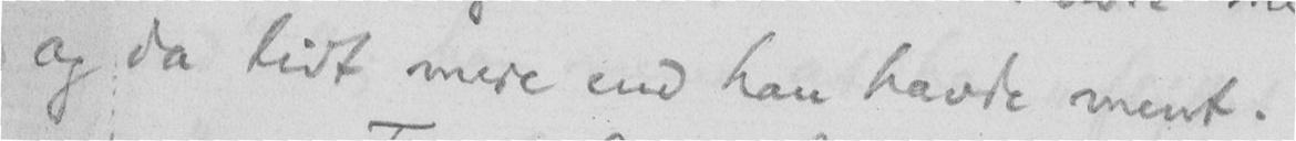og da lidt mere end han havde ment.
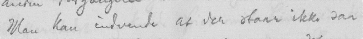Man kan indvende at der staar ikke saa
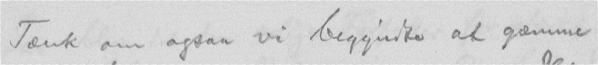Tænk om ogsaa vi begyndte at gæmme
